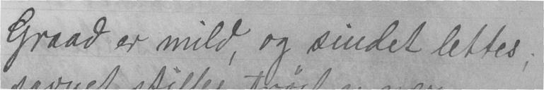Graad er mild, og sindet lettes;
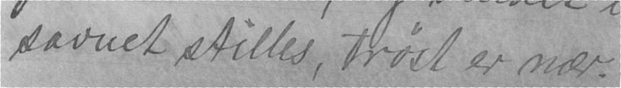savnet stilles, trøst er nær.
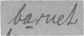barnet
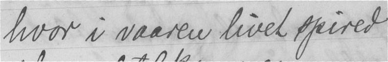hvor i vaaren livet spired
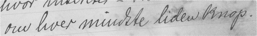om hver mindste liden knop.
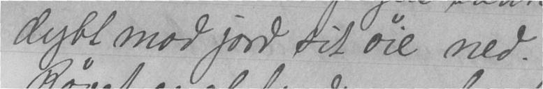dybt mod jord sit øie ned.
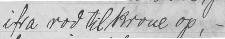ifra rød til krone op, -
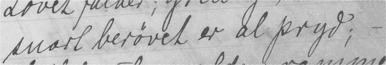snart berøvet er al pryd; -
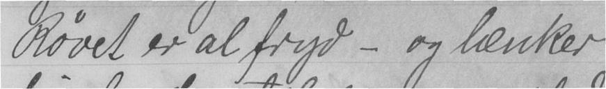Røvet er al fryd - og lænker
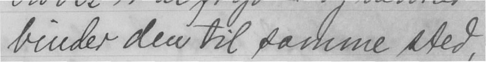binder den til samme sted,
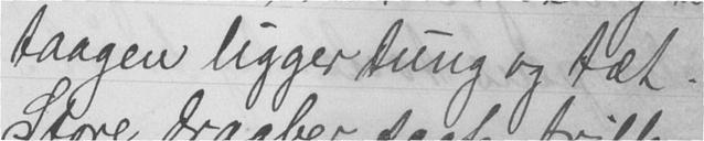taagen ligger tung og tæt.
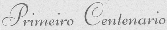Primeiro Centenario
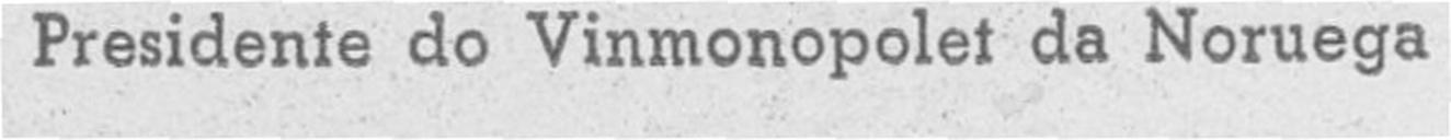Presidente do Vinmonopolet da Noruega
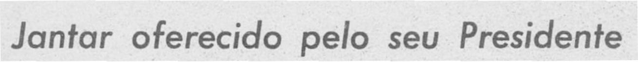Jantar oferecido pelo seu Presidente
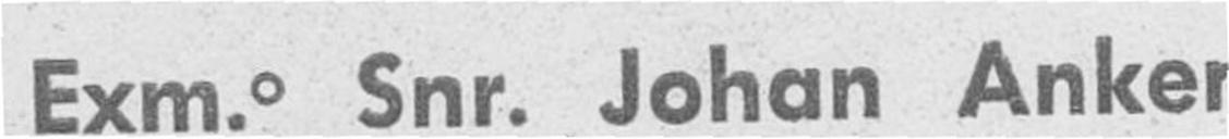Exm.° Snr. Johan Anker
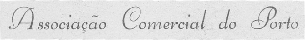Associacao Comercial do Porto
